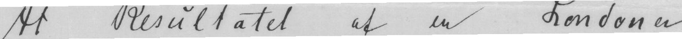At Resultatet af en Londoner
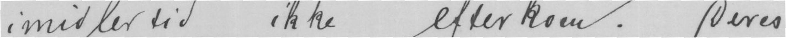imidlertid ikke efterkom. Deres
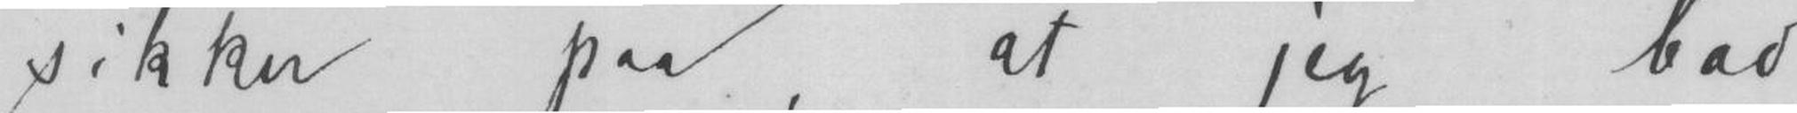sikker paa, at jeg bad
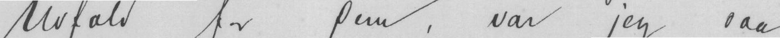udfald for dem, var jeg saa
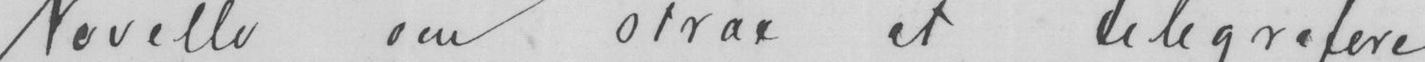Novelle som strax at telegrafere
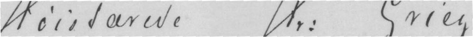Høistærede Hr. Grieg!
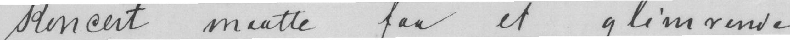koncert maatte faa et glimrende
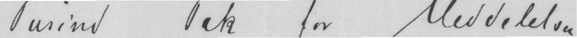Tusind Tak for Meddelelsen,
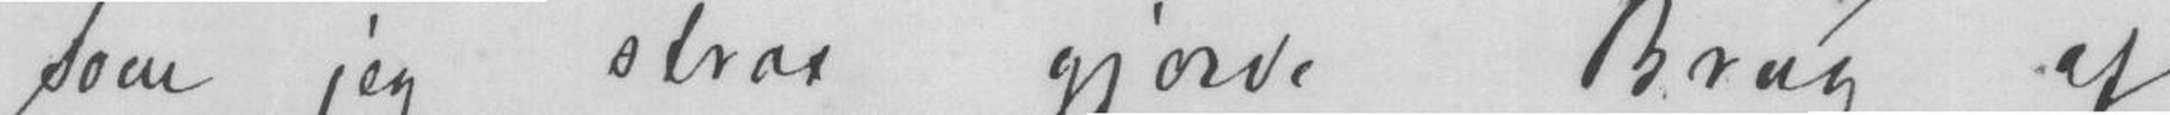som jeg strax gjorde Brug af.
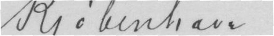Kjøbenhavn 12/5 88
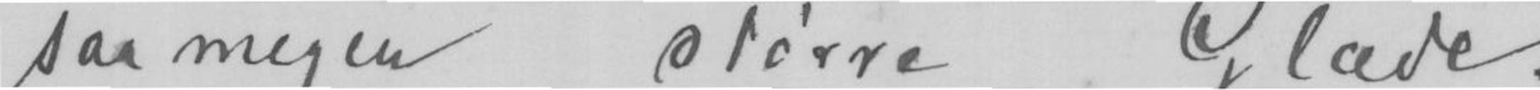saa meget større Glæde.
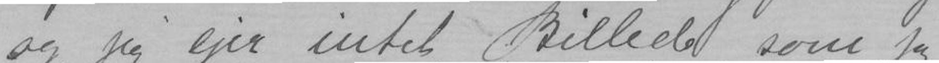og jeg ejer intet Billede, som jeg
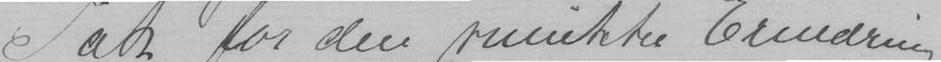Tak for den smukke Erindrimg
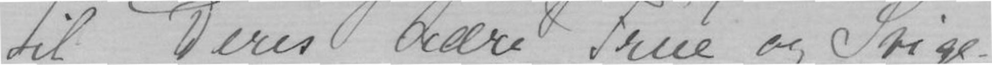til Deres kære Frue og Svige-
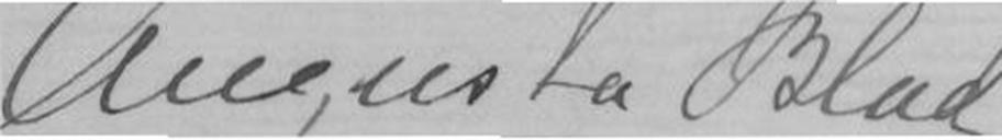Augusta Blad.
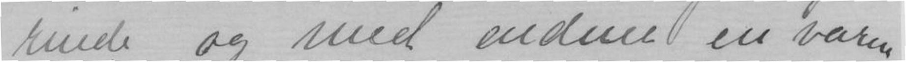rinde og med endnu en varm
